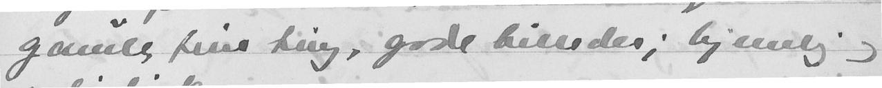gamle fine ting, gode billeder; hjemlig og
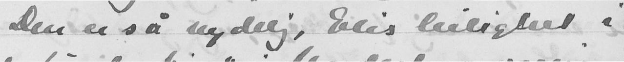Den er så nydelig, Elis leilighet i
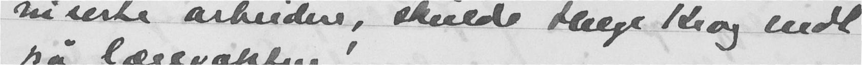niserte arbeidere, skulde Helge Krog endt
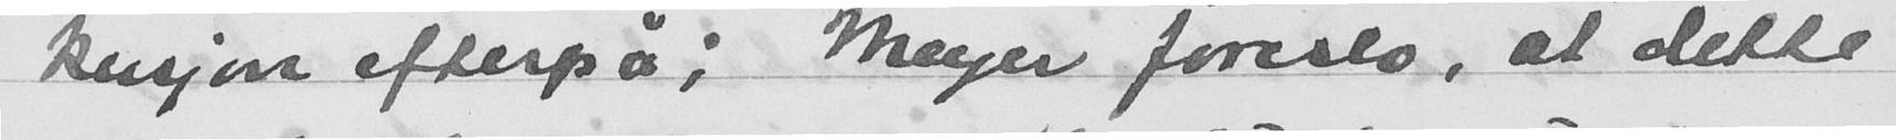kusjon efterpå; Meyer foreslo, at dette
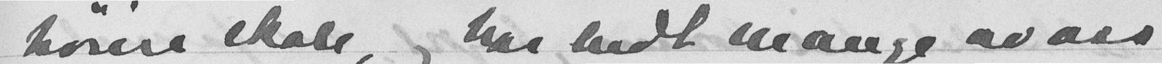høiere skole, og har bedt mange av oss
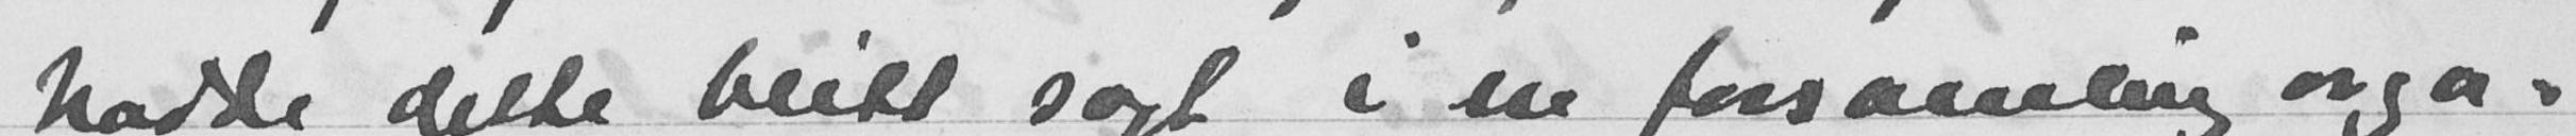hadde dette blitt sagt i en forsamling orga-
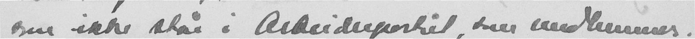som ikke står i Arbeiderpartiet som medlemmer.
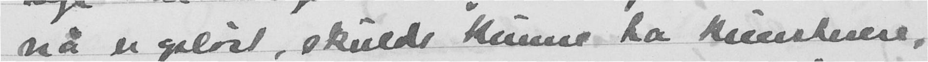nå er opløst, skulde kunne ha kunstnere,
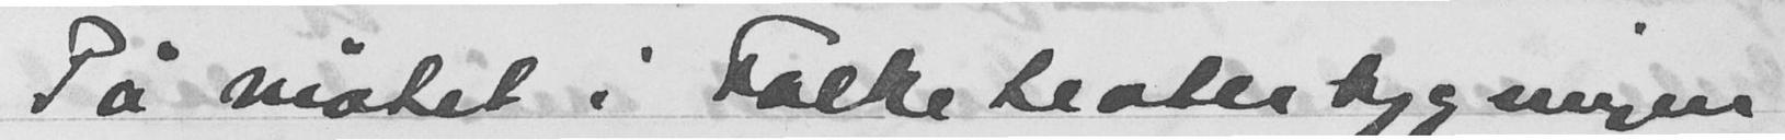På møtet i Folketeaterbygningen
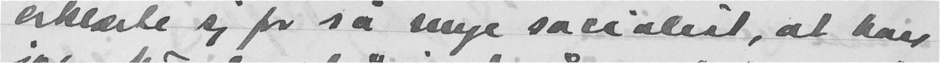erklærte sig for så mye socialist, at han
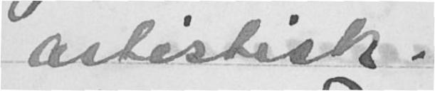artistisk.
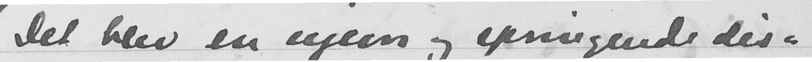Det blev en ujevn og springende dis-
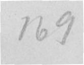169
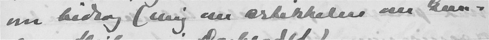om bidrag (mig om ærtikkelens om Gun-
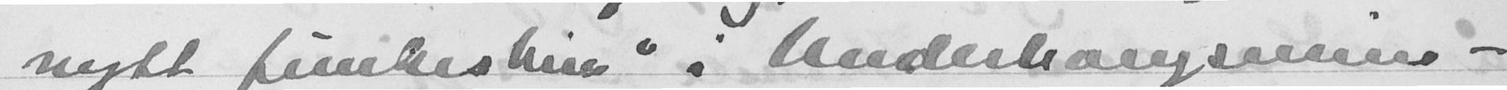nytt "funkishus" i Underhaugsveien -
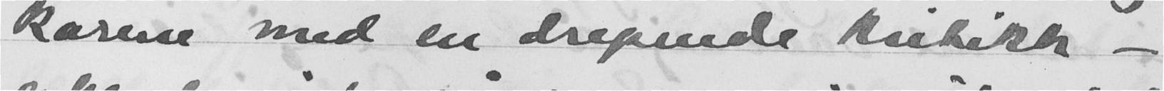karene med en drepende kritikk -
